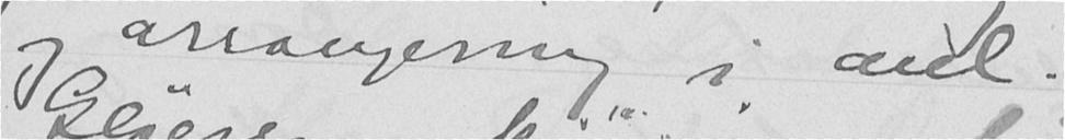og arrangering i anl.
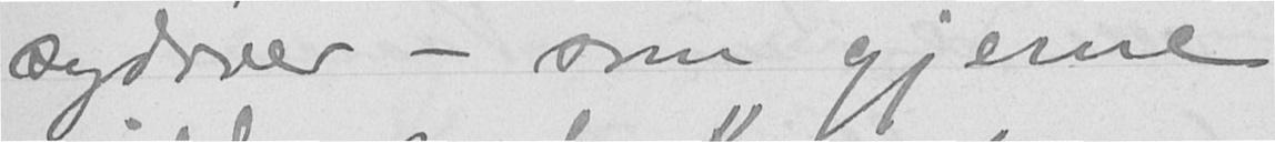sydover - som gjerne
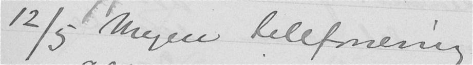12/5 Megen telefonering
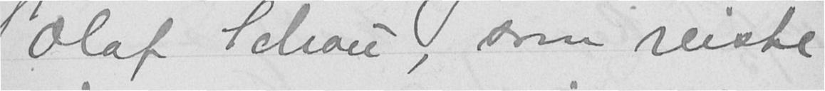Olaf Schou, som reiste
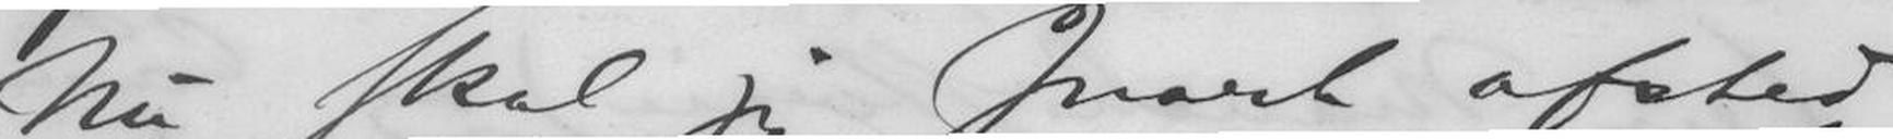Nu skal jeg snart afsted
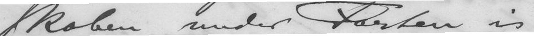skaben under Farten i
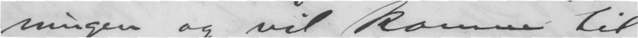ningen og vil komme til
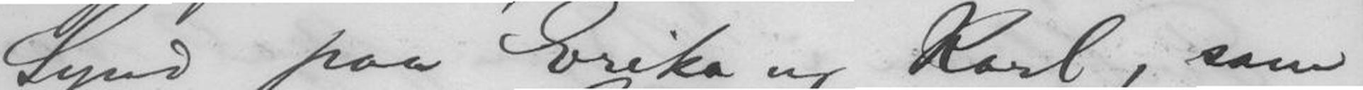Synd paa Erika og Karl, som
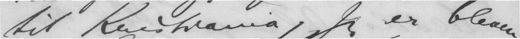til Kristiania. Jeg er bleven
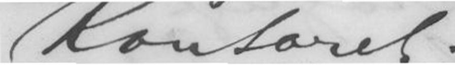Kontoret.
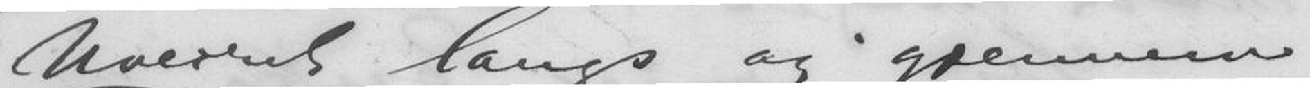Uveiret langs og gjennem
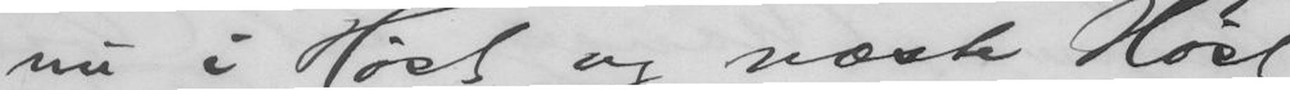nu i Høst og næste Høst
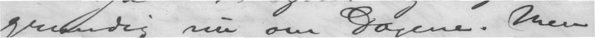grundig nu om Dagene. Men
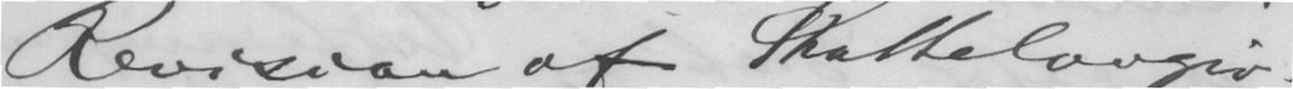Revision af Skattelovgiv-
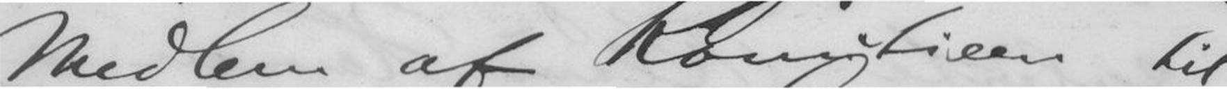Medlem af Komitieen til
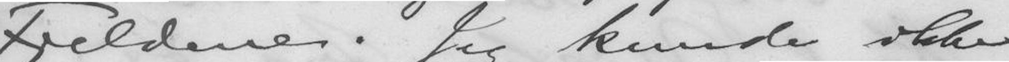Fjeldene. Jeg kunde ikke
